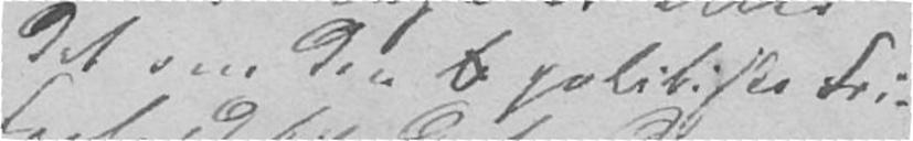det om den politiske Fri-
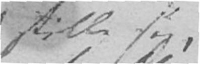stille sig,
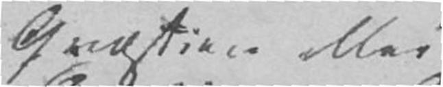Qvæstion eller
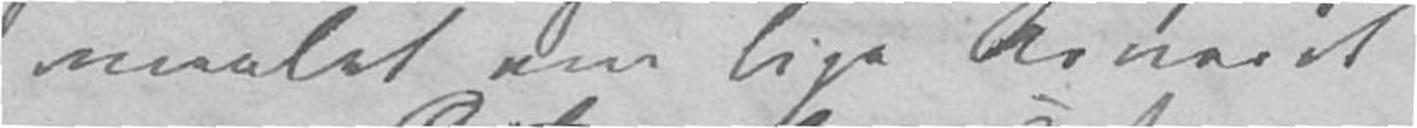maalet om lige Arveret
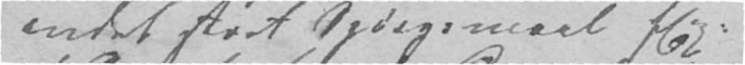andet stort Spørgsmaal fEx:
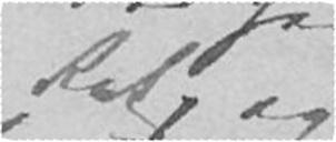Ret, og
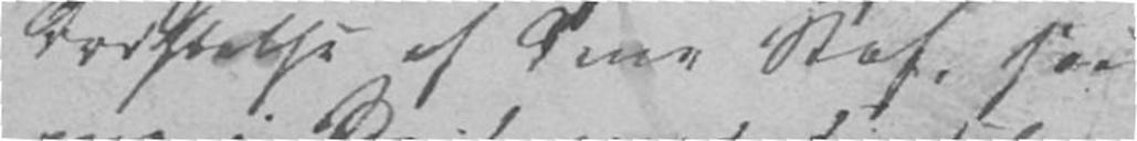Drøftelse af dens Stof, saa
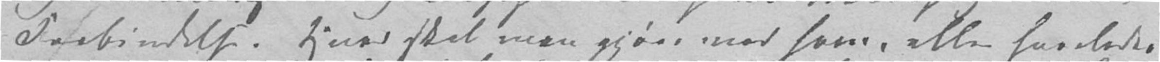Forbindelse. Hvad skal man gjøre med ham, eller hvorledes
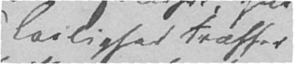Leilighed træffer
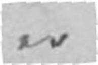er
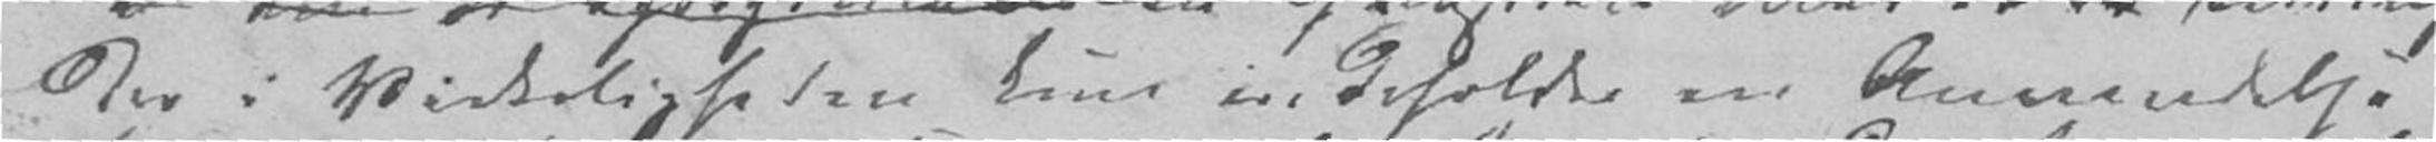der i Virkeligheden kun indeholder en Anvendelse
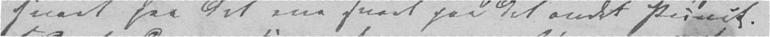snart paa det ene snart paa det andet Punct.
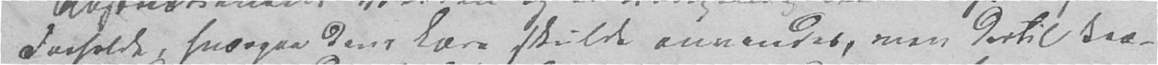Forholde, hvorpaa dens Lære skulde anvendes, men dertil kræ-
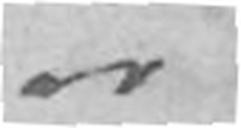er
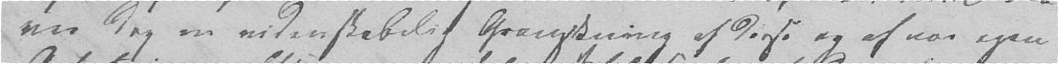ves dog en videnskabelig Granskning af disse og af vor egen
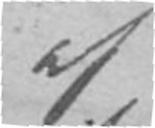af
